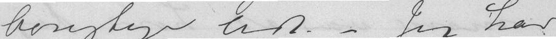besigtege lidt. Jeg har
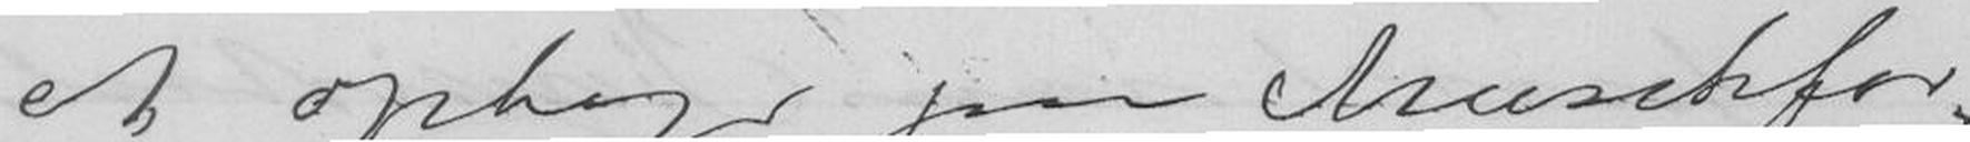at optage paa Musikfor-
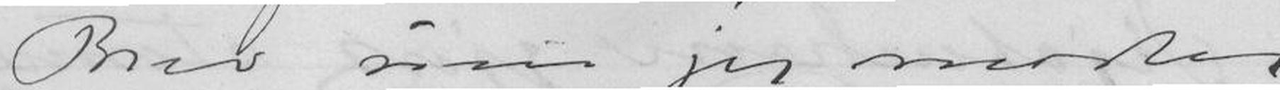Brev som jeg modtog
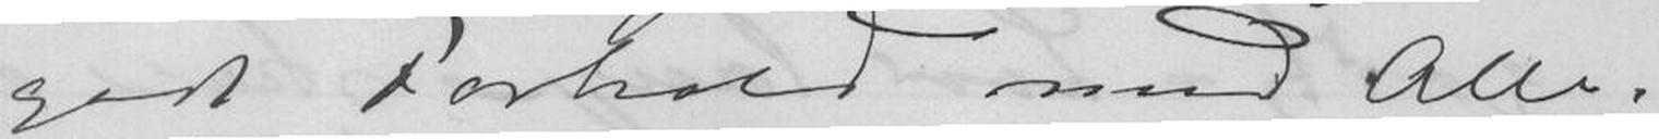godt Forhold med Alle.
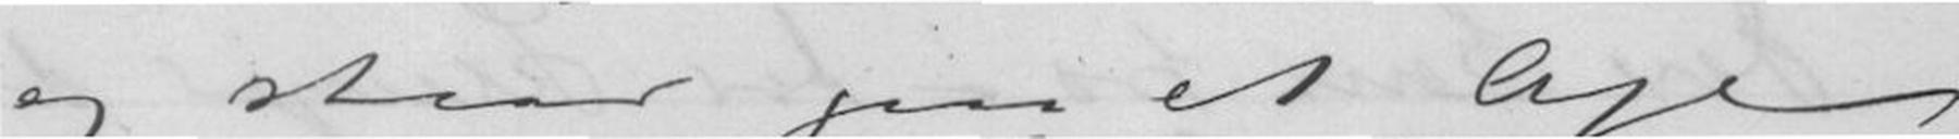og staar paa et lige
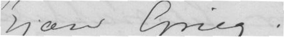Kjære Grieg!
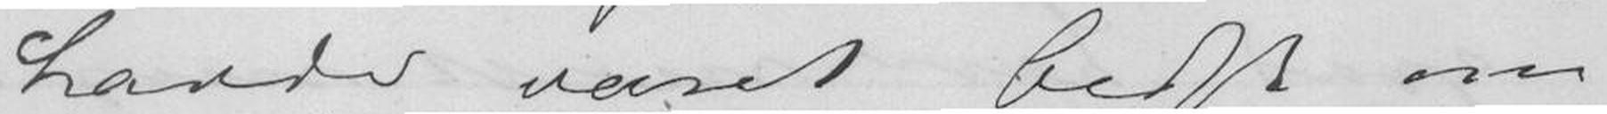havde været bedst om
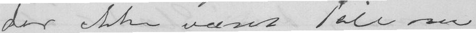der ikke været Tale om
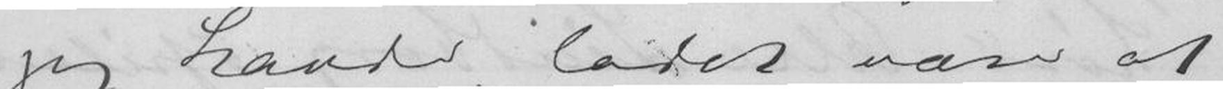jeg havde ladet være at
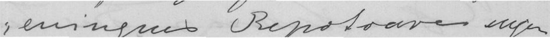eningens Reportoares ingen
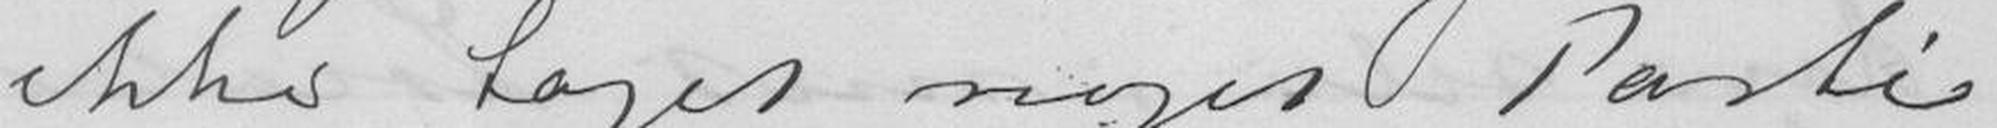ikke taget noget Parti
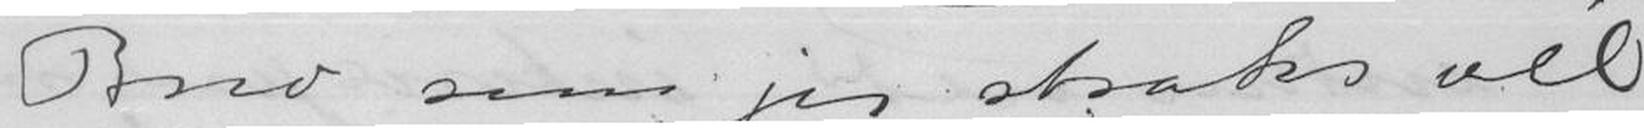Brev som jeg straks vil
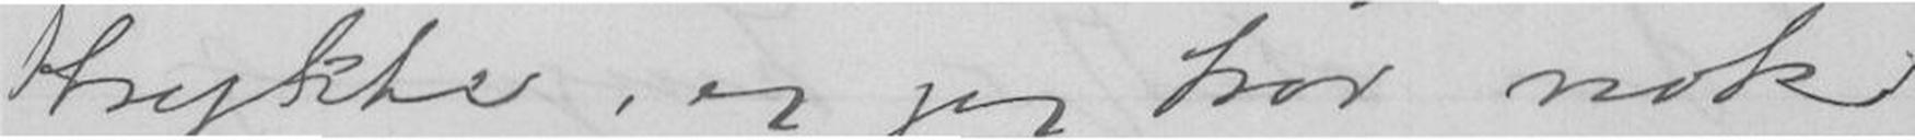trykte, og jeg tror nok
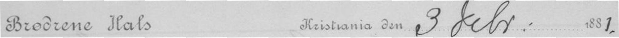Brødrene Hals Kristiania den 3 Dcbr. 1881
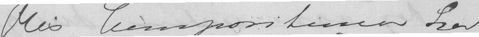Oles kompositioner har
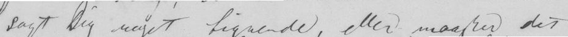sagt Dig noget lignende, eller maaske det
